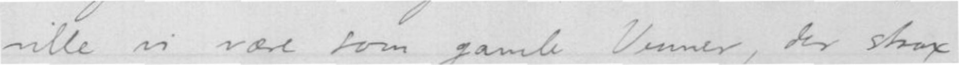ville vi være som gamle Venner, der strax
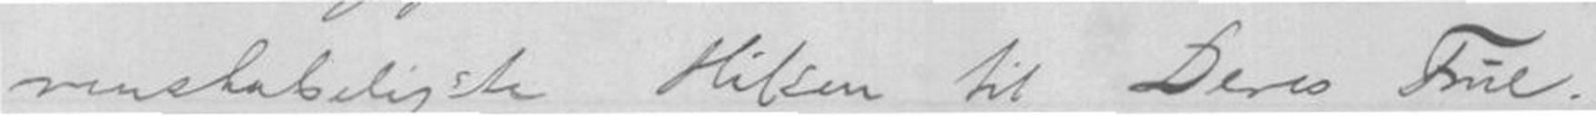beligste hilsen til Deres Frue.
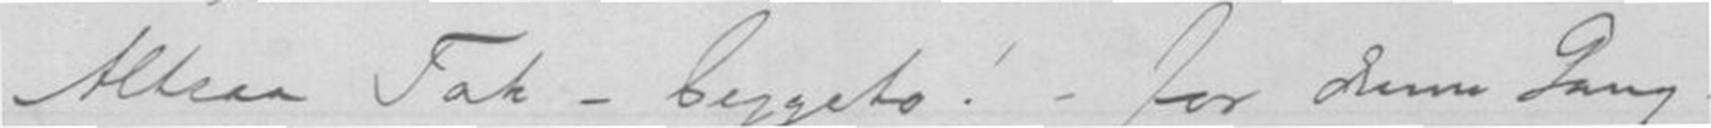Altsaa Tak - beggeto! for denne Gang
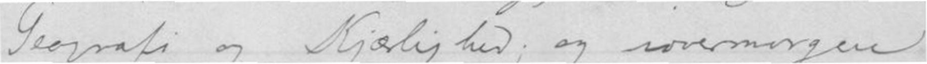Geografi og Kjærlighed; og iovermorgen
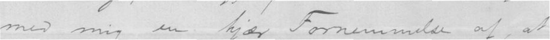med mig en kjær Fornemmelse af, at
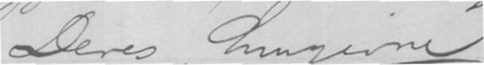Deres Hengivne
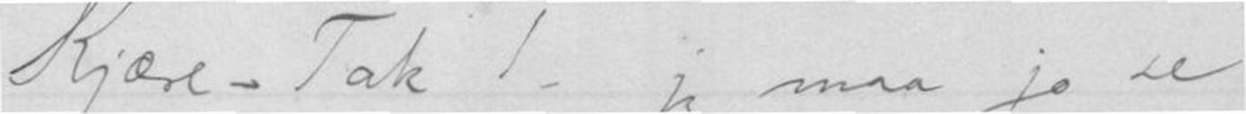Kjære Tak 1. Jeg maa jo se
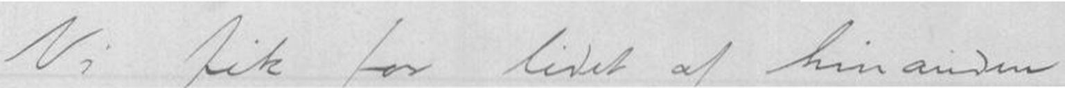Vi fik for lidet af hinanden
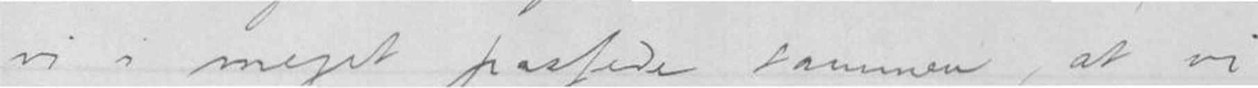vi i meget pasfede sammen, at vi
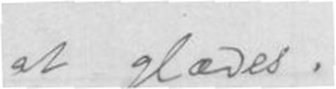at glædes.
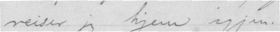reiser jeg hjem igjen.
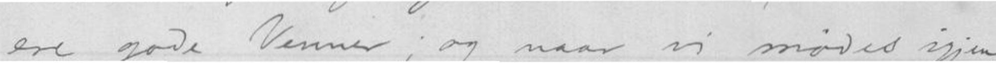ere gode Venner; og naar vi mødes igjen
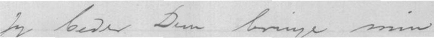Jeg beder Dem bringe min
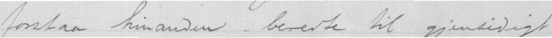forstaar hinanden beredte til gjensidigt
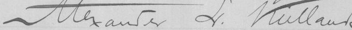Alexander L. Kielland
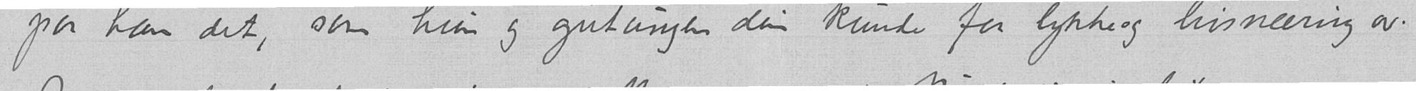paa ham det, som hun og gutungen din kunde faa lykke og livsnæring av.
